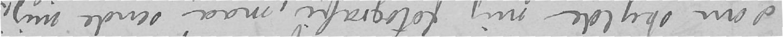som skylde mig fotografi, maa sende mig,
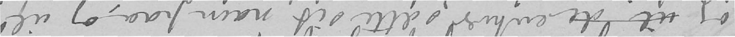og vil de enhver sætte sit navn paa, og vil
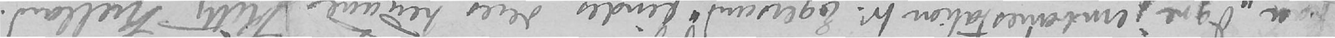paa «Ogne jernbanestation pr. Egersund» findes deres hengivne Kitty Kielland.
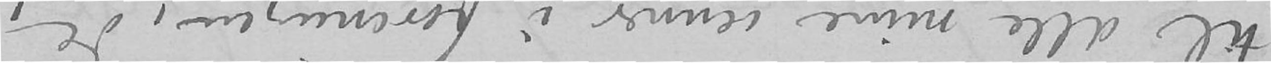til alle mine venner i foreningen, de,
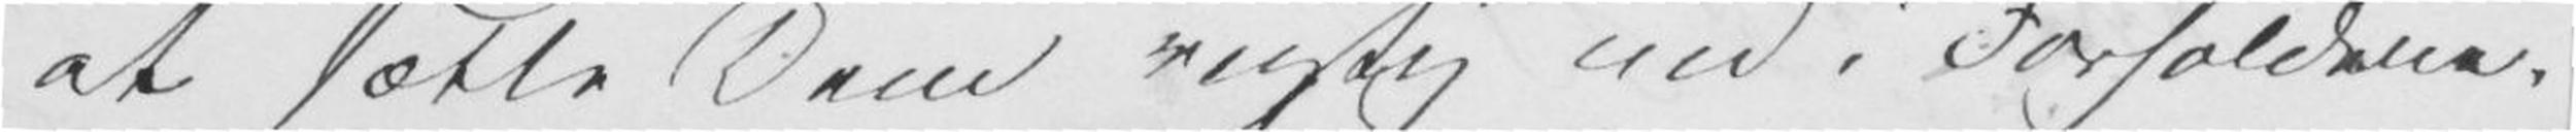at sætte Dem rigtig ind i Forholdene.
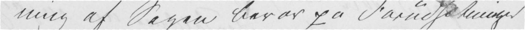ning af Sagen berør på Forudsætninger
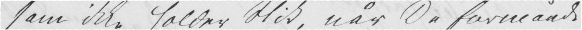som ikke holder Stik, når De formåede
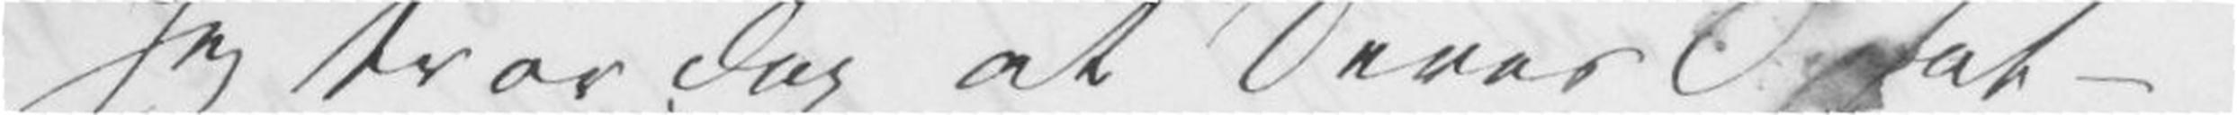Jeg tror dog at Deres Opfat¬
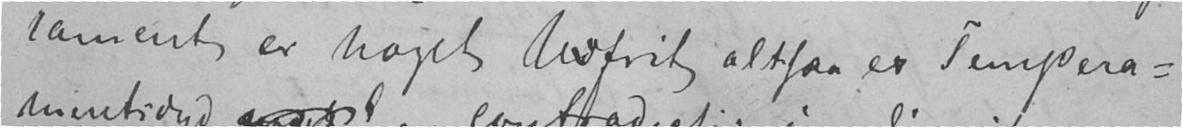rament er noget Ufrit altsaa er Tempera-
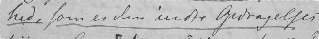hed, som er den indre Opdragelses
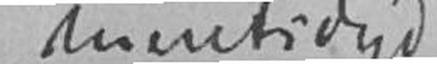mentsdyd
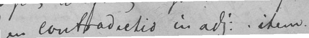en Contradiction in adj: item.
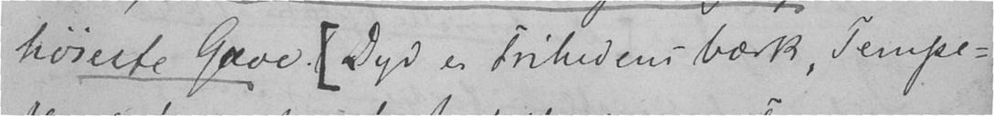høieste Gave [Dyd er Frihedens Værk, Tempe-
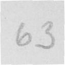63
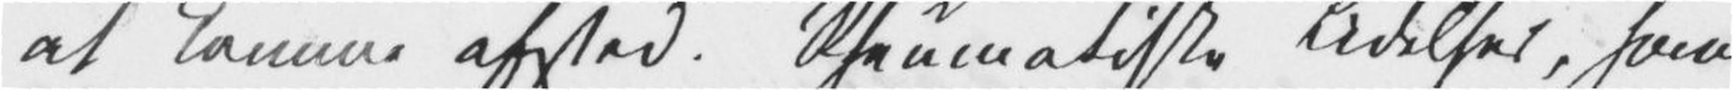at komme afsted. Rheumatiske Lidelser, som
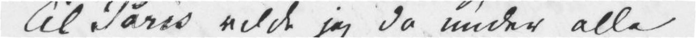Til Paris vilde jeg da under alle
